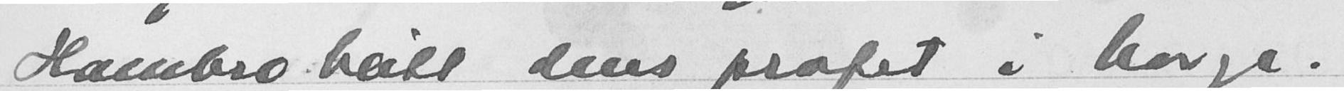Hambro blitt dens profet i Norge.
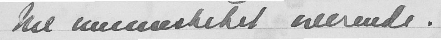hel menneskehet overende.
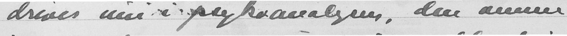drives inn i psykoanalysen, den annen
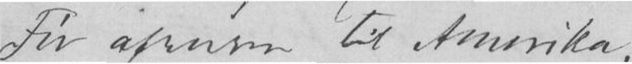Før afreisen til Amerika,
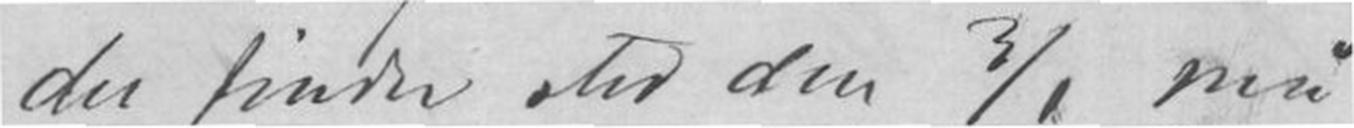der findes sted den 3/1 må
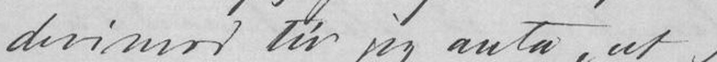derimod til jeg anta , ut og
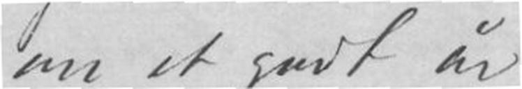nu et godt år.
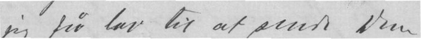jeg få lov til at sende Dem
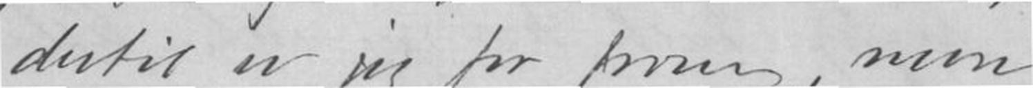dertil er jeg for men
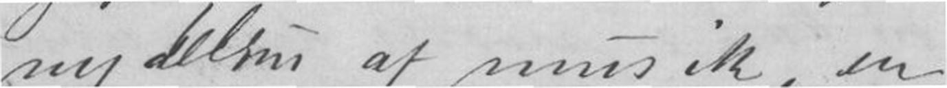nydelse af musik, en
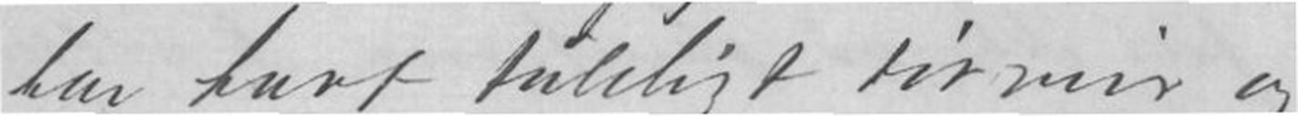har kavt taleligt og
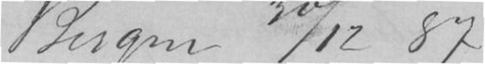Bergen 30/12 87
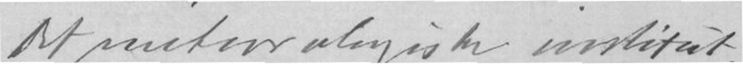Det meteorologiske institut,
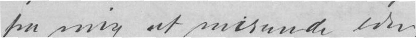fra mig at misunde eder,
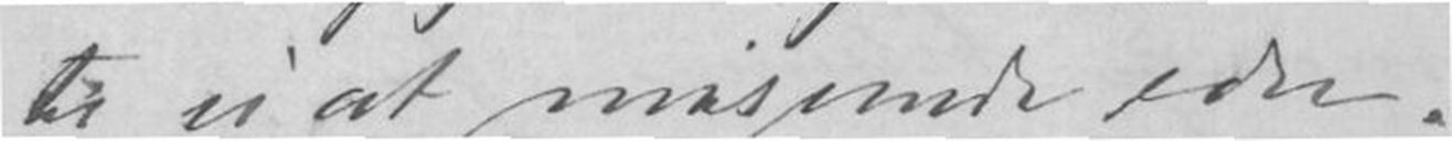til ei at misunde eder.
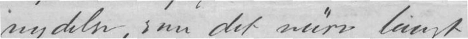nydelse som det langt
